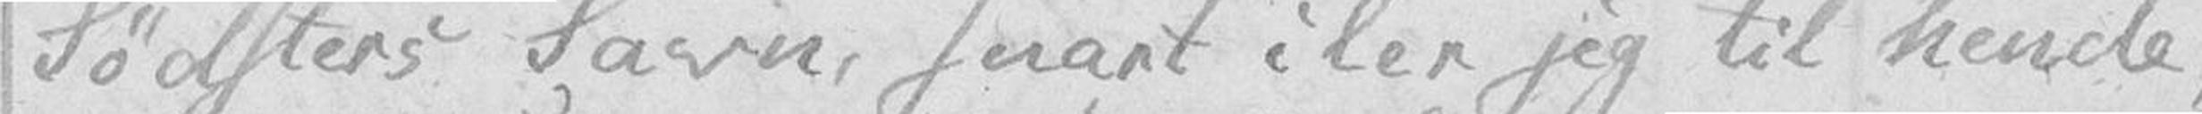Sødsters Savn, snart iler jeg til hende,
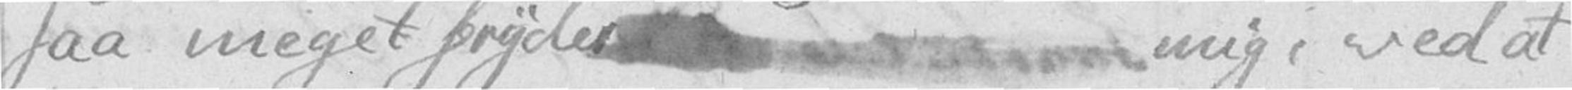saa meget fryder  mig, ved at
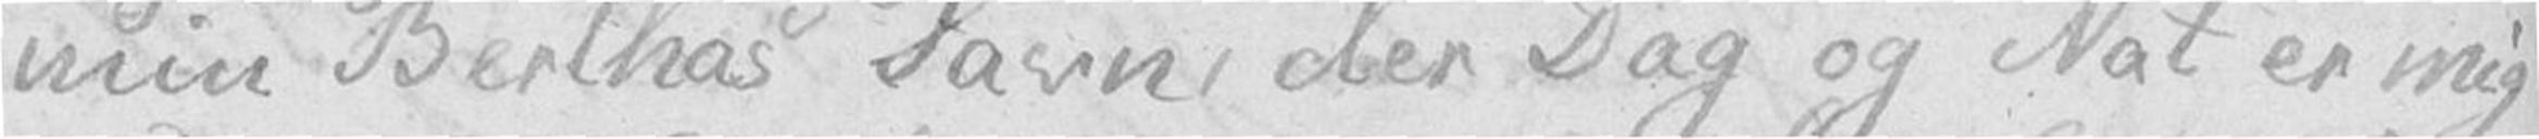min Berthas Savn, den Dag og Nat er mig
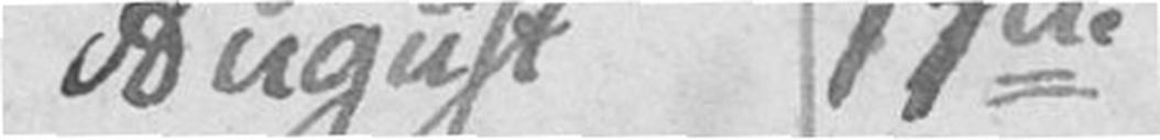August 17de
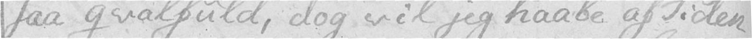saa qvalfuld, dog vil jeg haabe af Tiden
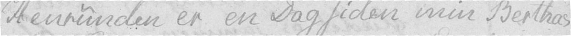Henrunden er en Dag siden min Berthas
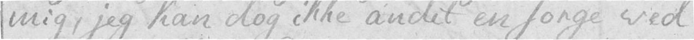mig, jeg kan dog ikke andet en sorge ved
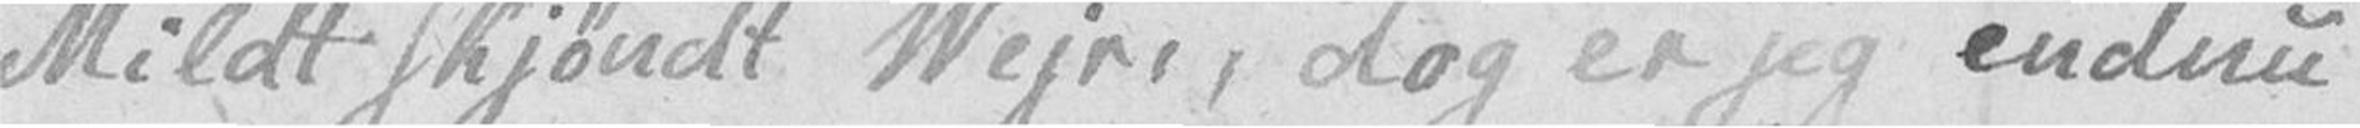Mildt skjøndt Vejr, dog er jeg endnu
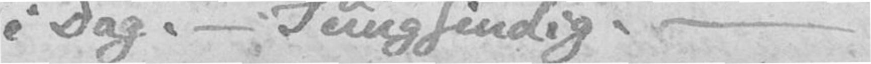i Dag. – Tungsindig. –
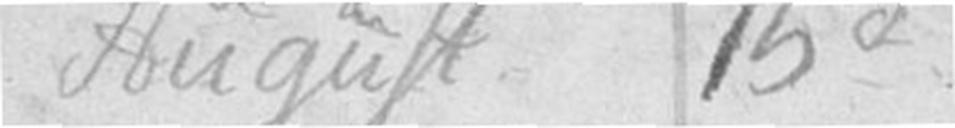August 15d
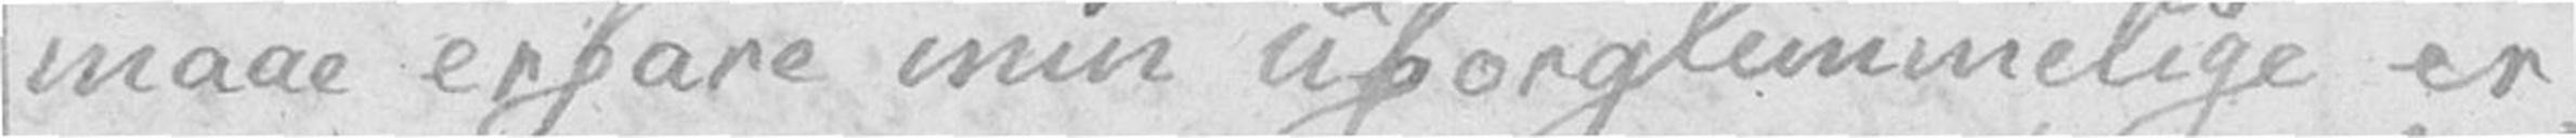maae erfare min uforglemmelige er
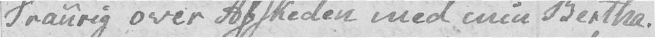Traurig over Afskeden med min Bertha.
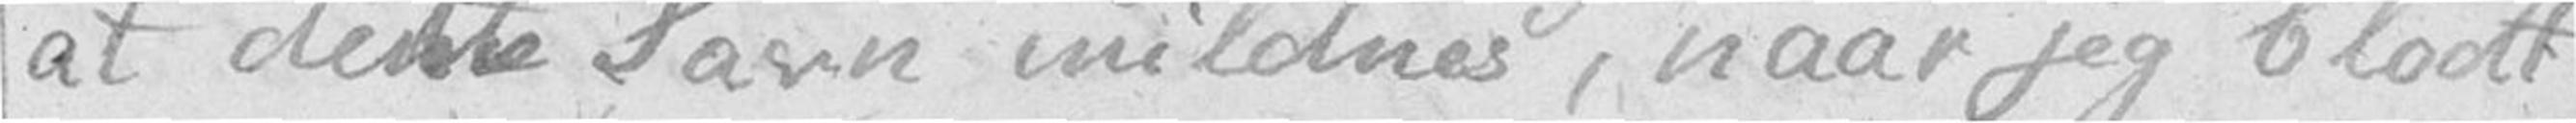at dette Savn mildnes, naar jeg blodt
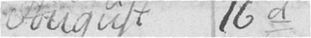August 16d
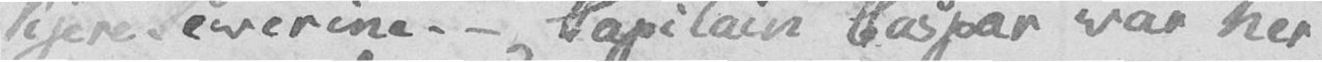kjere Severine. – Capitain Caspar var her
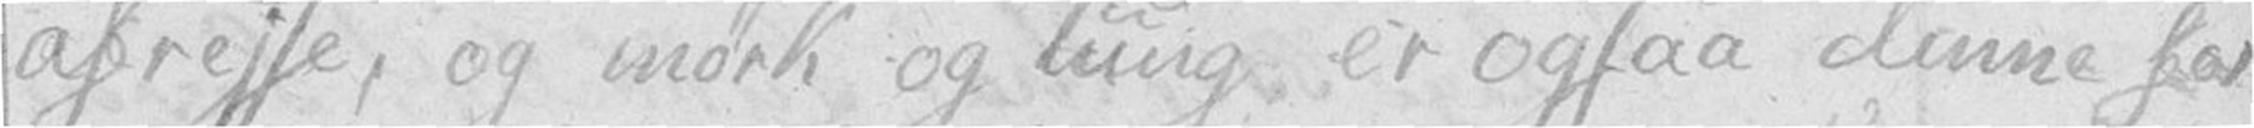afrejse, og mørk og tung er ogsaa denne for
